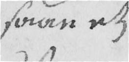saae et
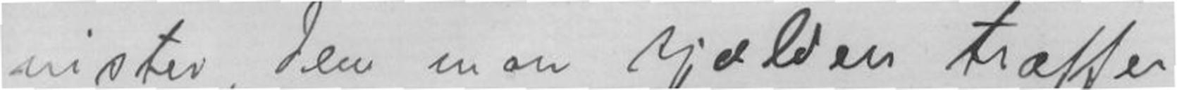nister, dem man sjælden træffer,
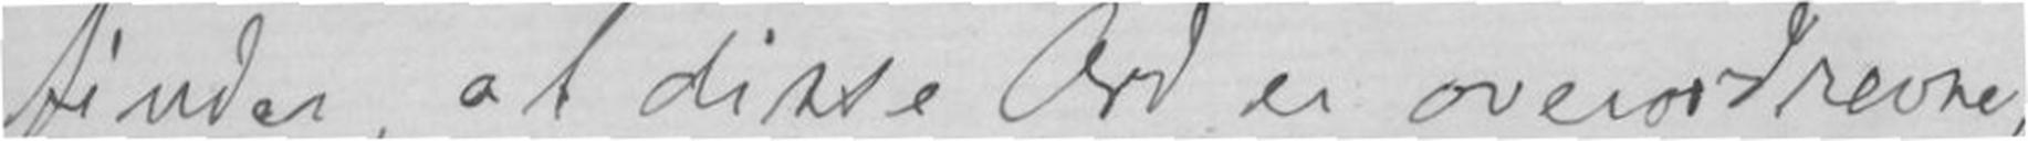finder, at disse Ord er overdrevne,
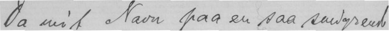Da mit Navn paa en saa svedgrende
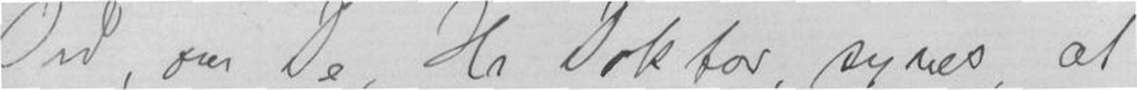Ord, om De, Hr Doktor, synes, at
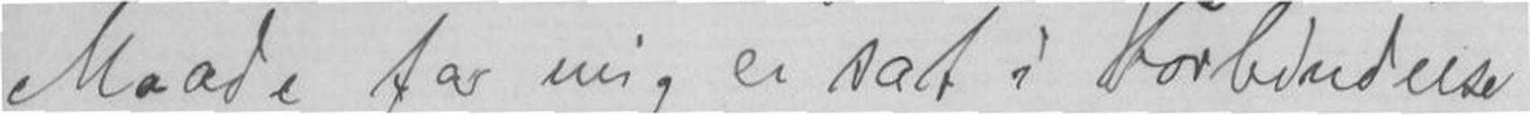Maade for mig er sat i Forbindelse
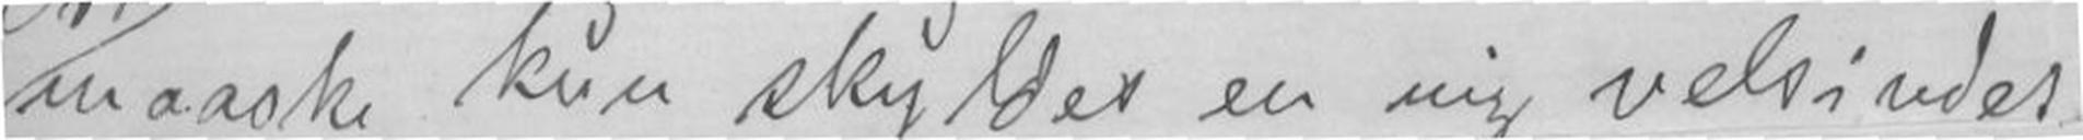Ord maaske kun skyldes en mig velsindet
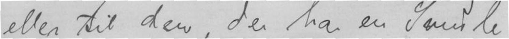eller til dem, der har en Smule
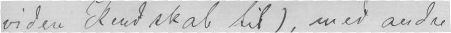videre Kundskab til), med andre
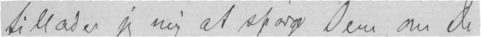tillader jeg mig at spørge Dem, om De
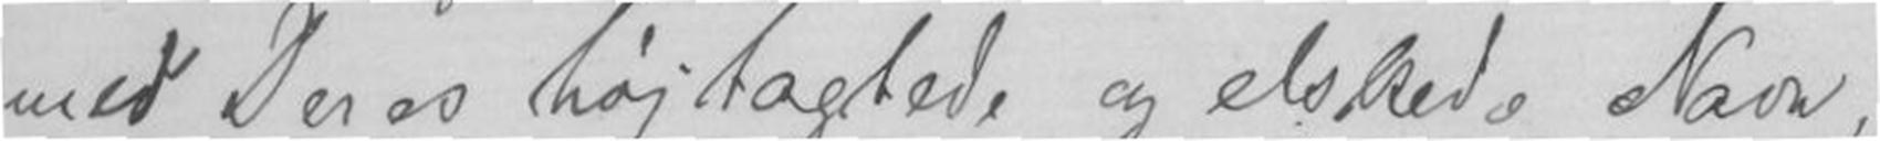med Deres højtagtede og elskede Navn,
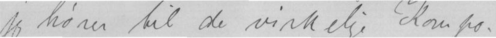jeg hører til de virkelige Kompo-
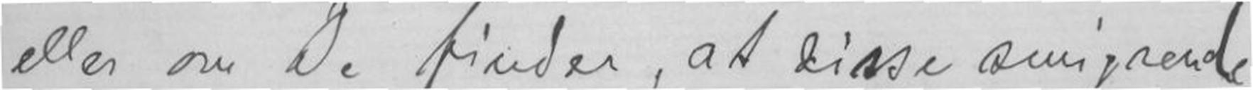eller om De finder, at disse smigrende
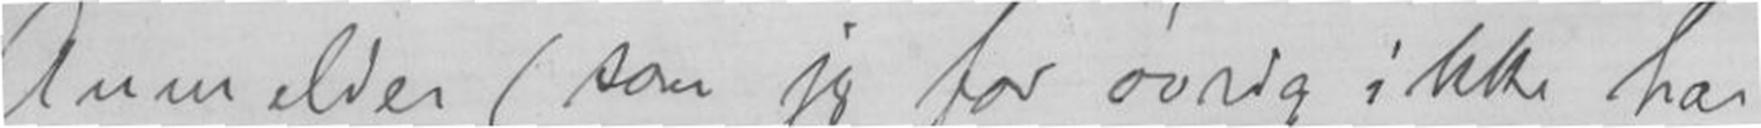Anmelder (som jeg for øvrig ikke har
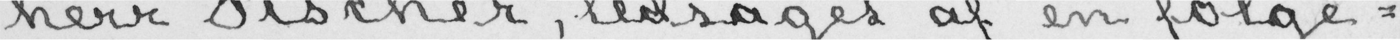herr Fischer, ledsaget af en følge-
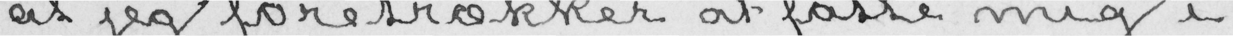at jeg foretrækker at fatte mig i
